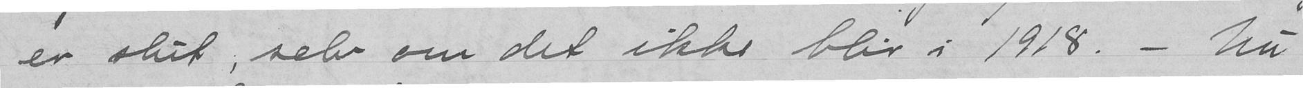er slut, selv om det ikke blir i 1918. - Nu
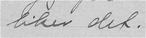liker det.
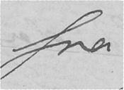fre
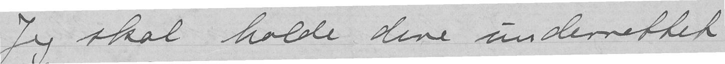Jeg skal holde dere underrettet
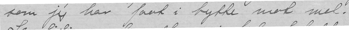som jeg har faat i bytte mot mel.
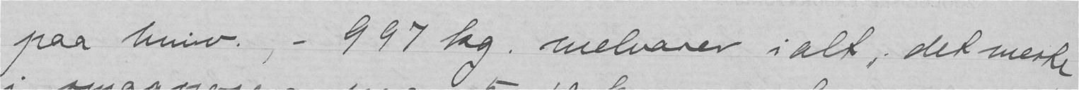paa $ - 997 kg. melvarer ialt, det meste
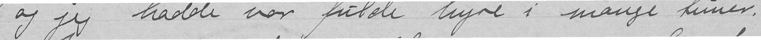og jeg hadde vor fulde hyre i mange timer.
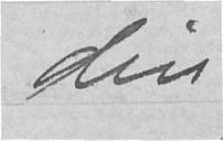din
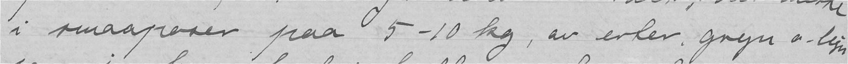i smaaposer paa 5-10 kg, år erter, gryn o-lign.
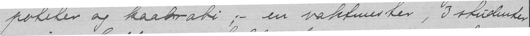poteter og kaalrabi ;- en vaktmester, 3 studenter
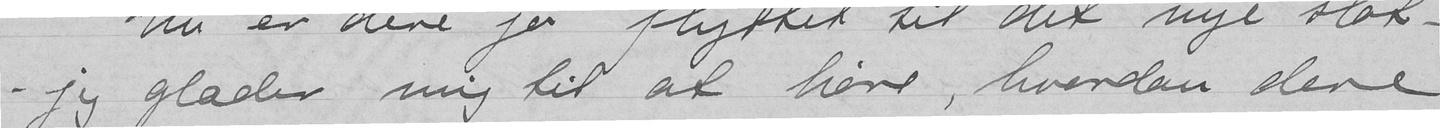- jeg glæder mig til at høre, hvordan dere
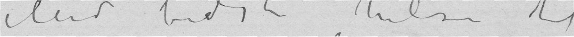Med bedste hilsen til
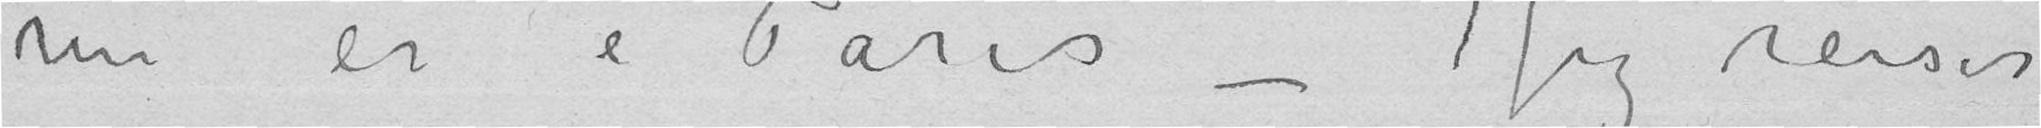nu er i Paris – Jeg reiser
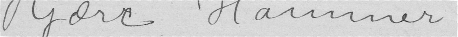Kjære Hammer!
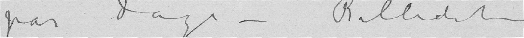par dage – Billedet
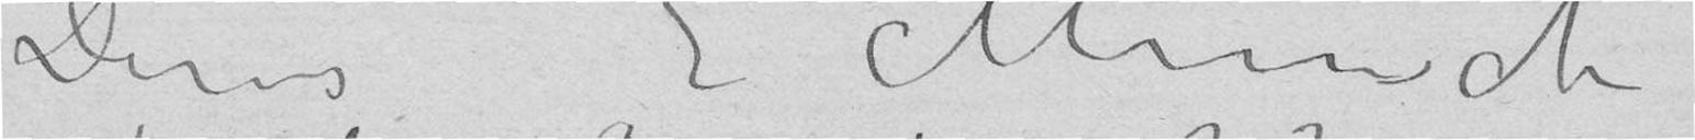Deres E Munch
